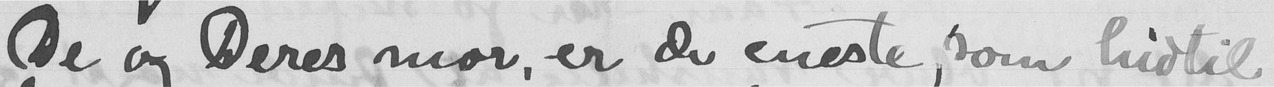De og Deres mor, er de eneste, som hidtil
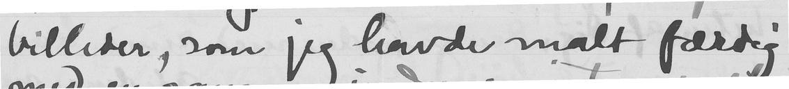billeder, som jeg havde malt færdig
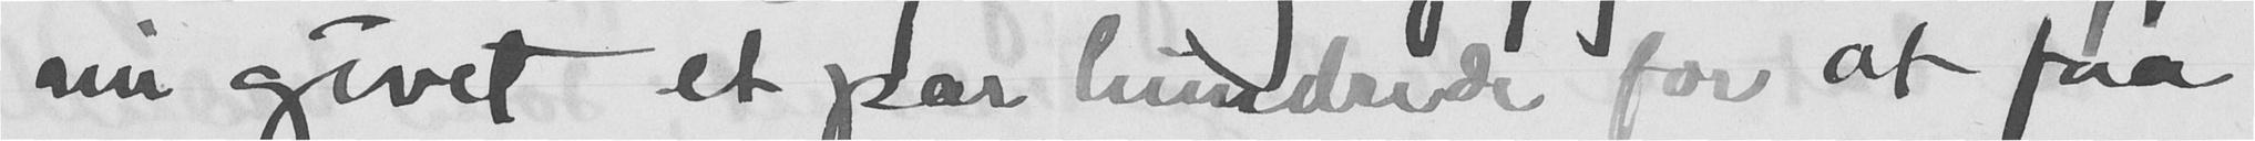nu givet et par hundrede for at faa
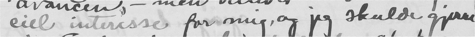ciel interesse for mig, og jeg skulde gjerne
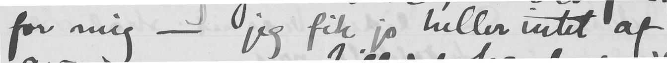for mig - jeg fik jo heller intet af
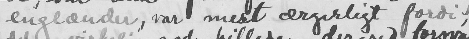englænder, var mest ærgerligt fordi,
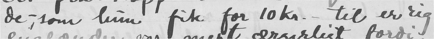de, som hun fik for 10 kr. - til en rig
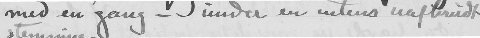med en gang -  under en intens uafbrudt
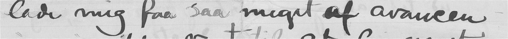lade mig faa saa meget af avancen -
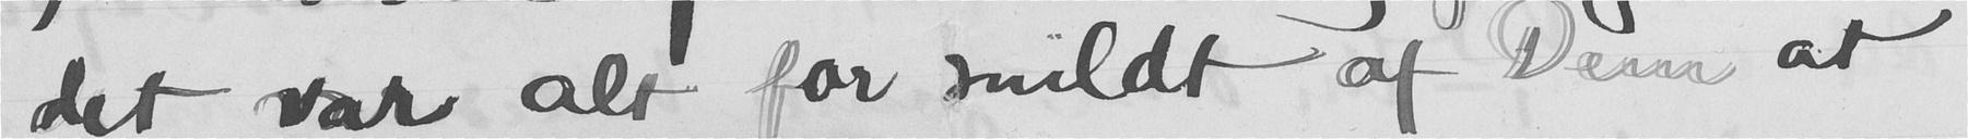det var alt for snildt af Dem at
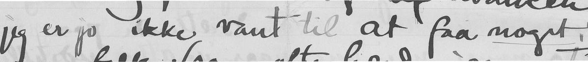jeg er jo ikke vant til at faa noget,
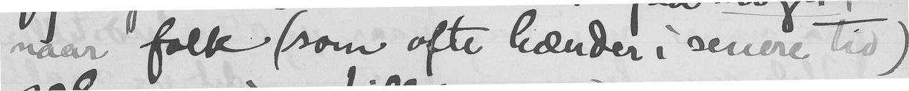naar folk (som ofte hænder i senere tid)
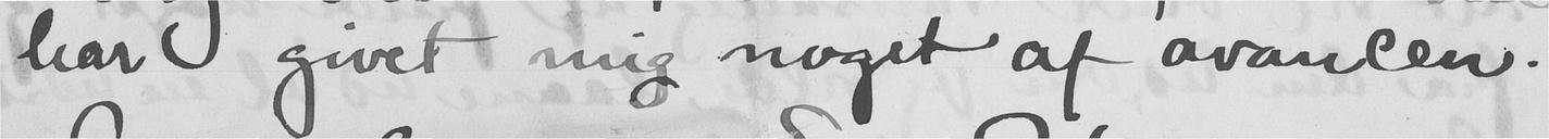har givet mig noget af avancen.
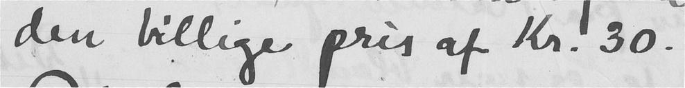den billige pris af Kr. 30.
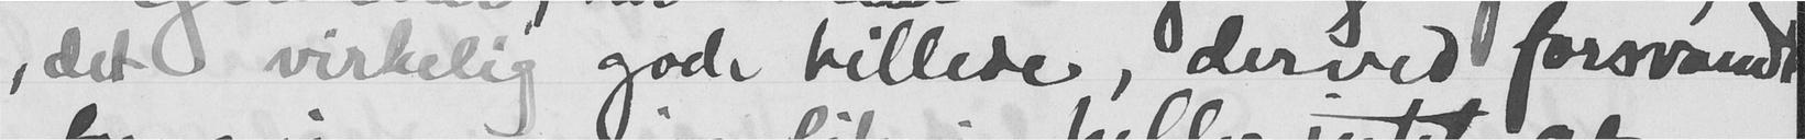, det virkelig gode billede, derved forsvandt
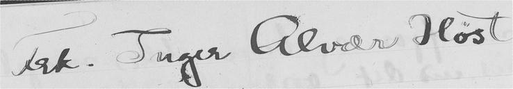Frk. Inger Alvær Høst
